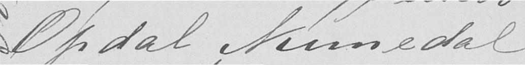Opdal Numedal
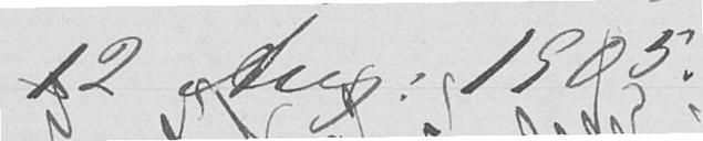12 Aug: 1905.
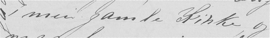i min gamle Kirke og
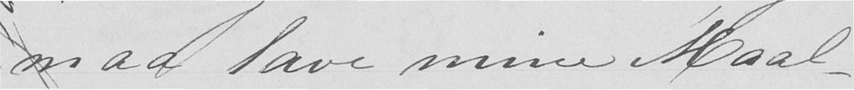maa lave mine Maal-
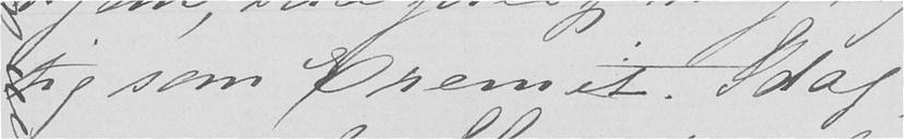tig som Eremit. Idag
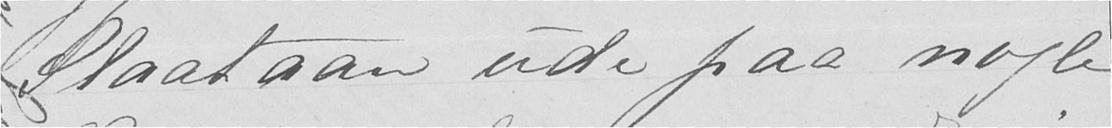Slaataan ude paa nogle
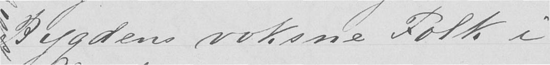Bygdens voksne Folk i
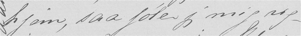hjem, saa føler jeg mig rig-
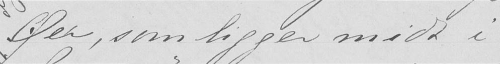Øer, som ligger midt i
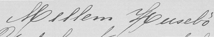Mellem Husebø
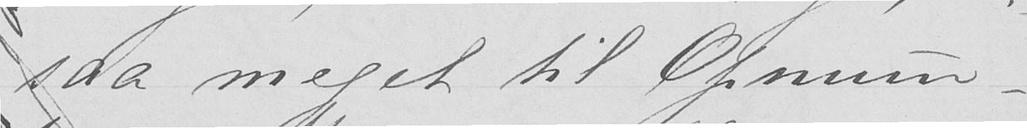saa meget til Opmun-
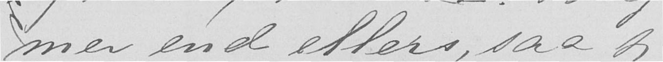mer end ellers, saa jeg
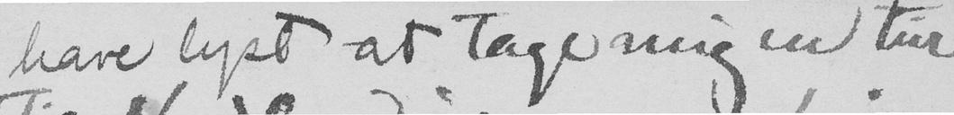have lyst at tage mig en tur
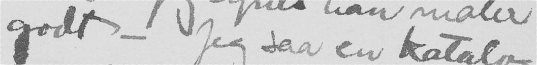godt - Jeg saa en katalog
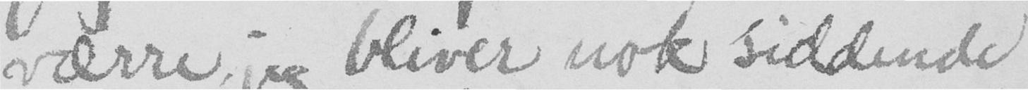værre, jeg bliver nok siddende
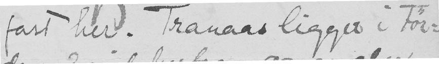fast her. Tranaas ligger i Før-
